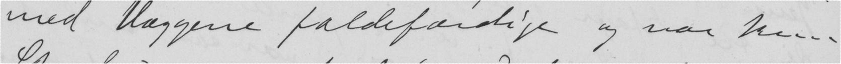med Væggene faldefærdige og var kun
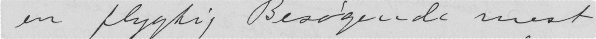en flygtig Besøgende mest
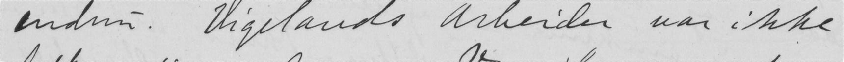endnu. Vigelands Arbeider var ikke
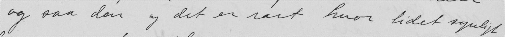og saa den og det er rart hvor lidet synligt
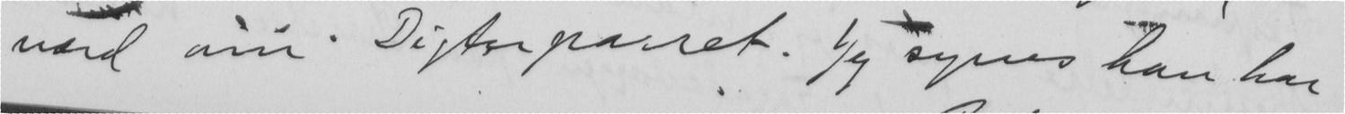værd om. Digterparret. Jeg synes han har
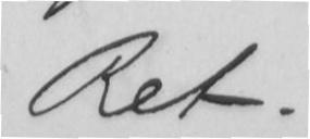Ret.
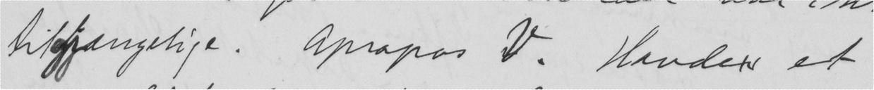tilgjængelige. Apropos V. Havde et
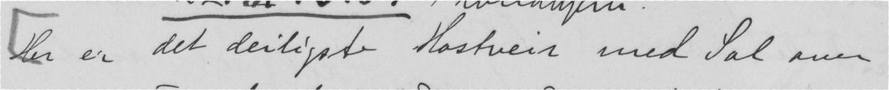Her er det deiligste Høstveir med Sol over
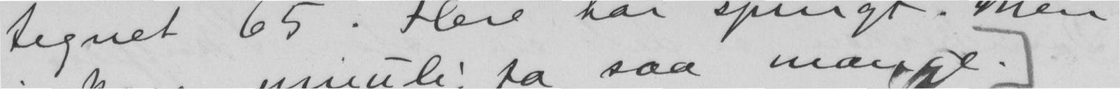tegnet 65. Flere har spurgt. Men
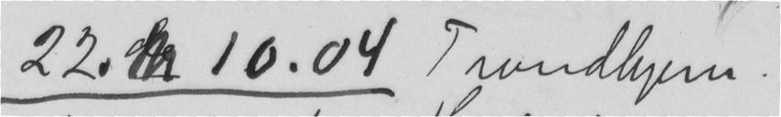22.  10.04 Trondhjem.
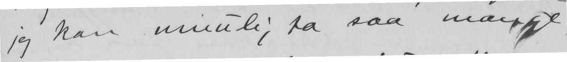jeg kan umulig ta saa mange.
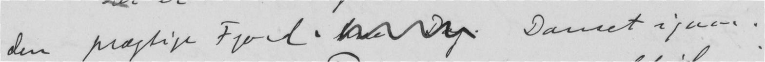den prægtige Fjord.  Danset igaar.
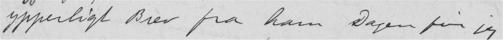ypperligt Brev fra ham Dagen før jeg
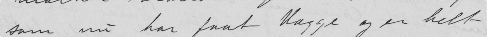som nu har faat Vægge og er helt
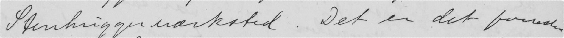Stenhuggerværksted. Det er det forresten
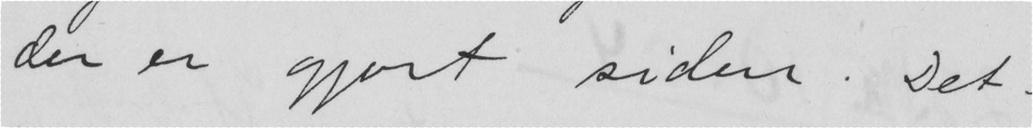der er gjort siden. Det
